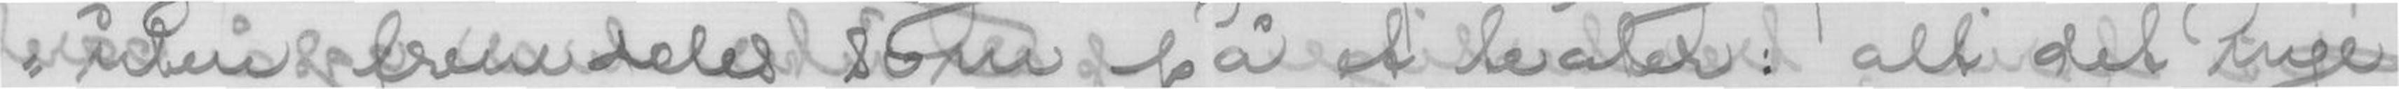uten fremdeles som på et teater; alt det nye
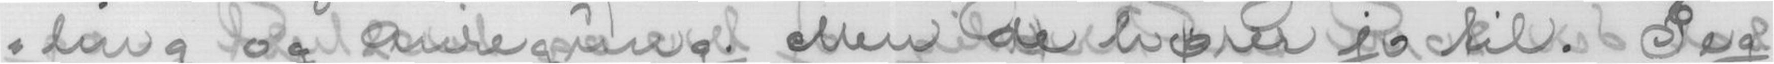ling og anregung. Men de hører jo til. Jeg
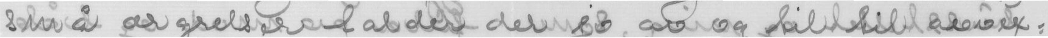små ærgrelser falder der jo av og til til avvex-
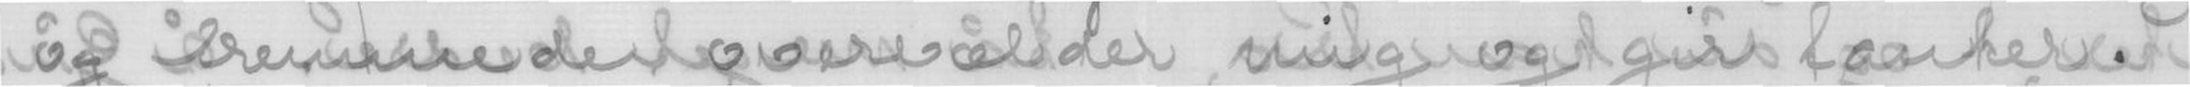og fremmede overvælder mig og gir tanker.
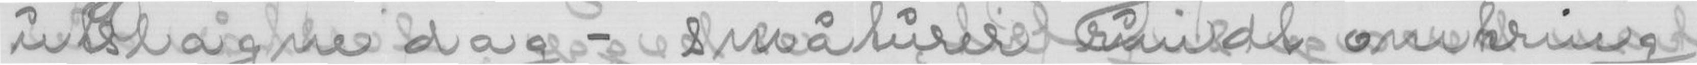utslagne dag - småturer rundt omkring
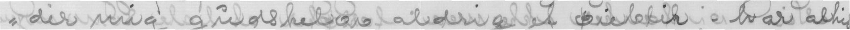der mig gudskelov aldrig et øieblik, - har altid
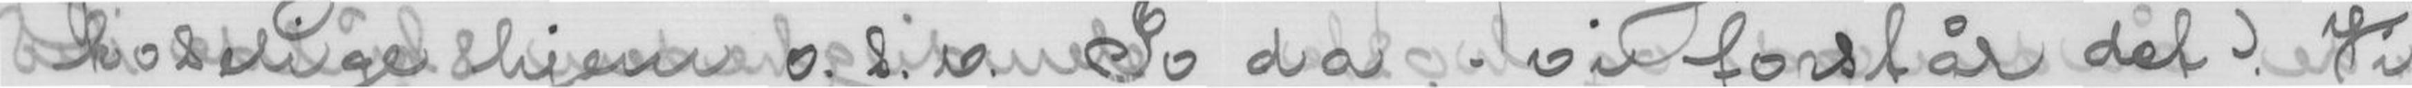koselige hjem o.s.v. Jo da, - vi forstår det! Vi
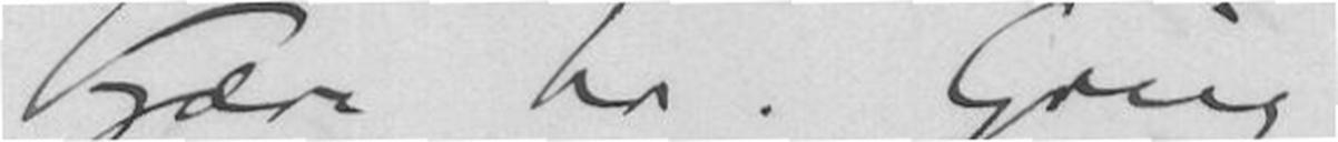Kjære hr. Grieg!
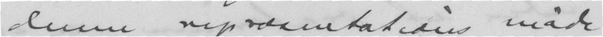denne repræsamtations måde
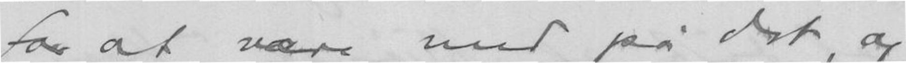for at være med på det, og
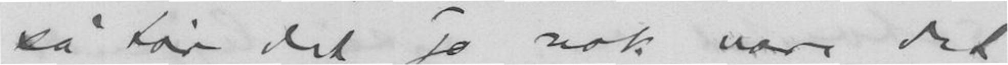så tør det jo nok være det
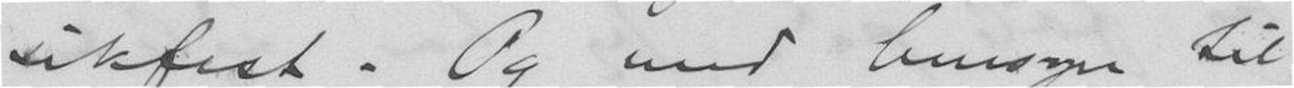sikfest. Og med hensyn til
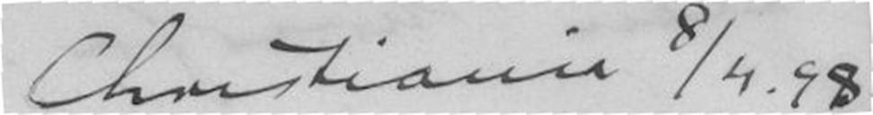Christiania 8/4.98
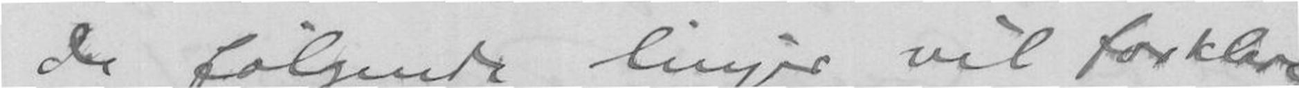de følgende linjer vil forklare
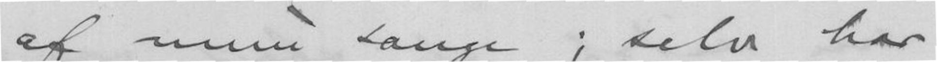af mine sange; selv har
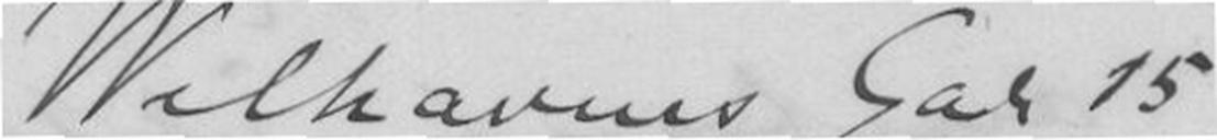Welhavner Gade 15
